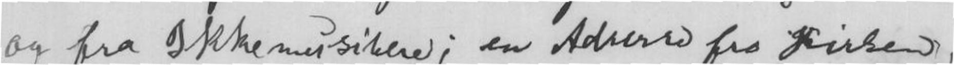og fra Ikke musikere; en Adresse fra Kirken,
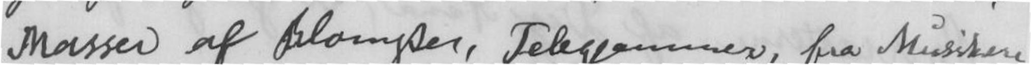Masser af Blomster, Telegrammer, fra Musikere,
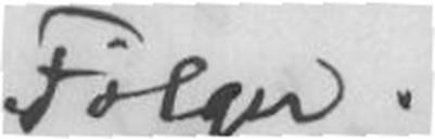Følger.
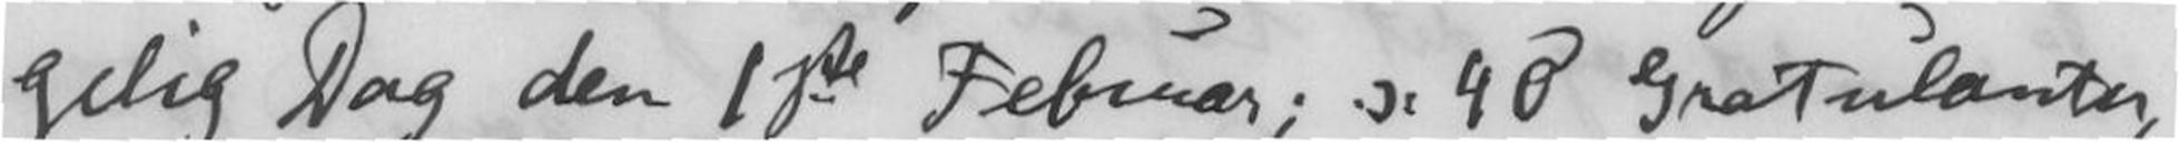gelig Dag den 1ste Februar; ɔ: 40 Gratulanter,
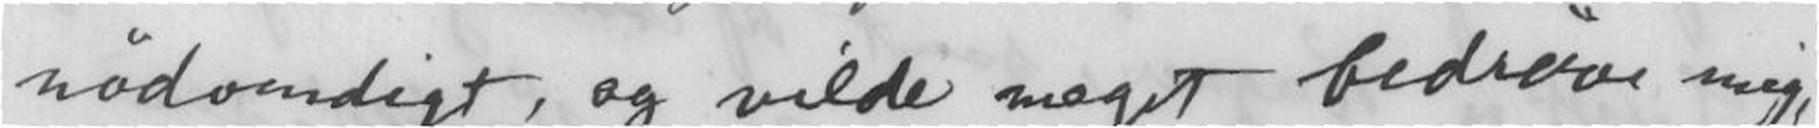nødvendigt, og vilde meget bedrøve mig,
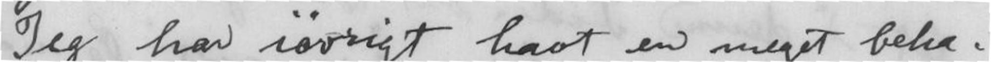Jeg har iøvrigt havt en meget beha-
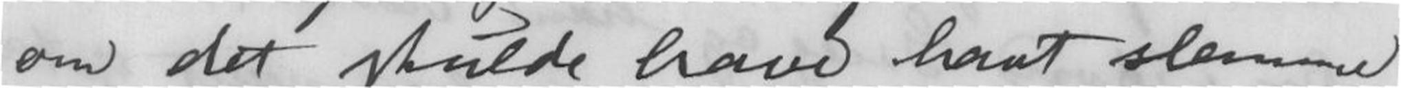om det skulde have havt slemme
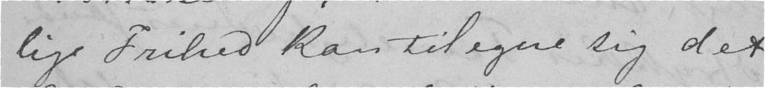lige Frihed kan tilegne sig det
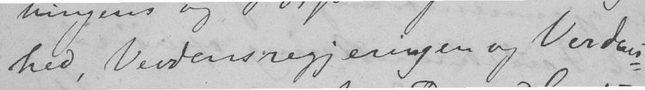hed, Verdensregjeringen og Verdens-
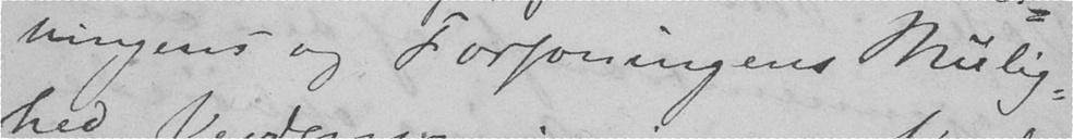ningens og Forsoningens Mulig-
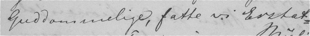Guddommelige, fatte vi Erstat-
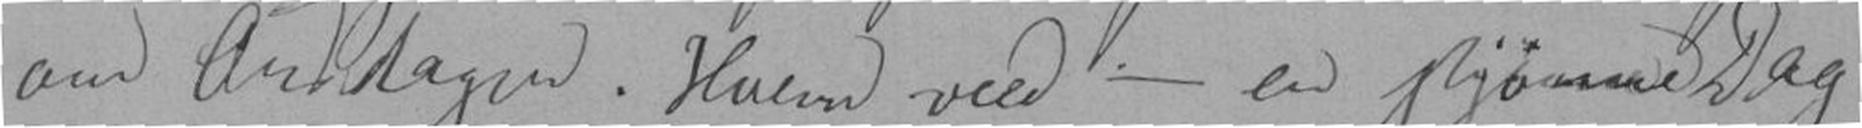om Onsdagen. Hvem veed - en skjønne Dag
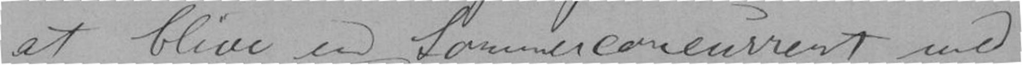at blive en Sommerconcurrent med
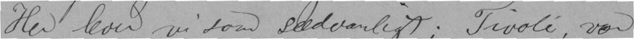Her lever vi som sædvanligt; Tivoli, vor
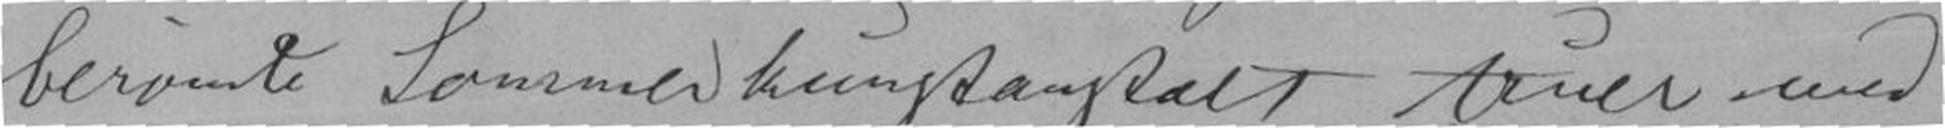berømte Sommerkunstanstalt truer med
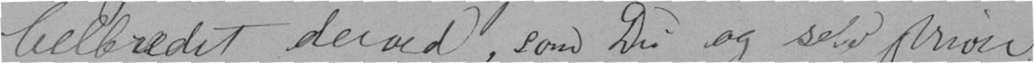helbredet derved, som Du og selv skriver,
